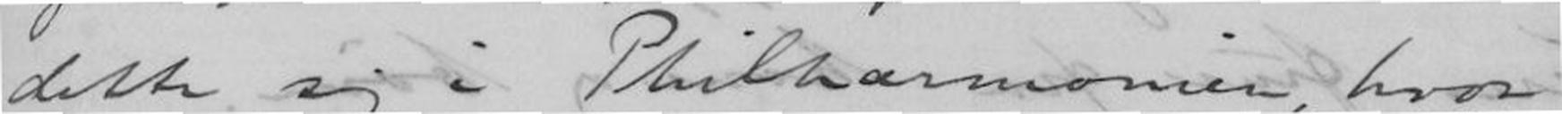dette sig i Philharmonien, hvor
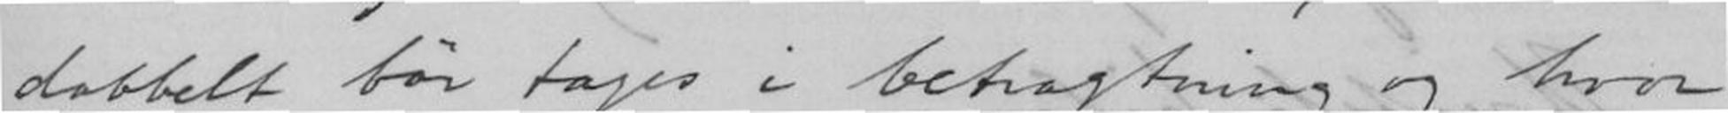dobbelt bør tages i betragtning og hvor
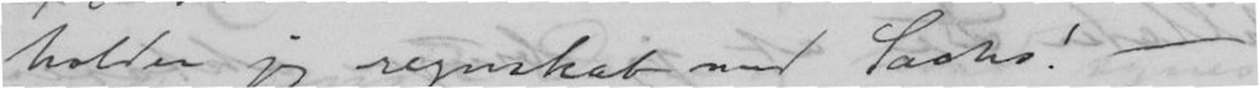holder jeg regnskab med Sachs! -
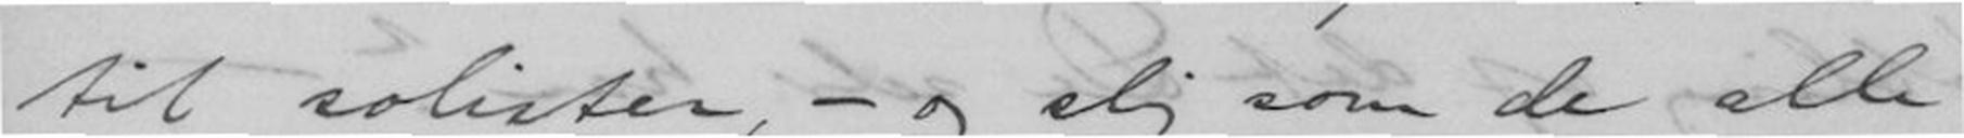til solister, - og slig som de alle
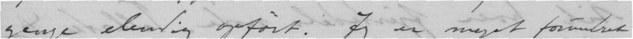gange elendig opført. Jeg er meget forundret
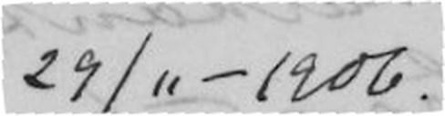29/11-1906
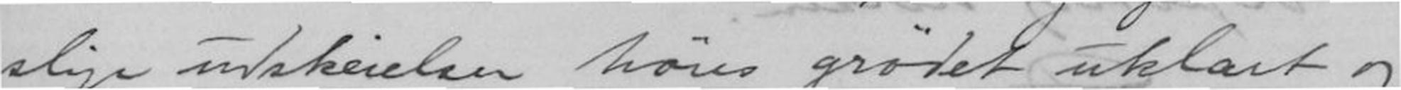slige udskeielser høres grødet uklart og
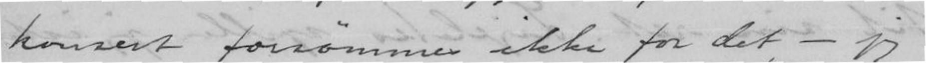konsert forsømmes ikke for det, - jeg
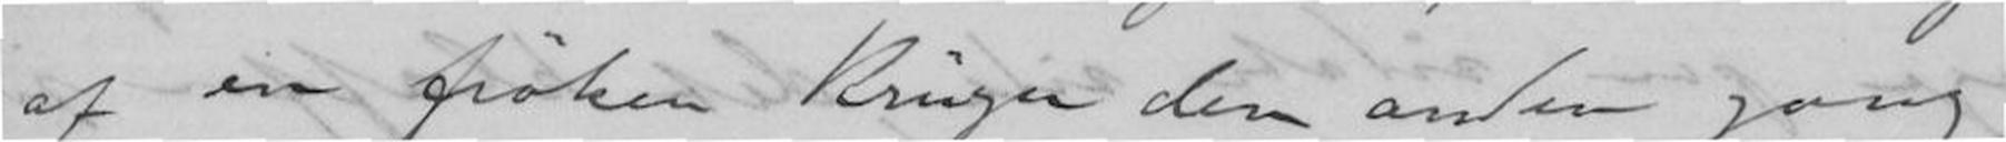af en frøken Krüger den anden gang
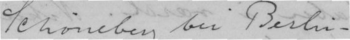Schöneberg bei Berlin
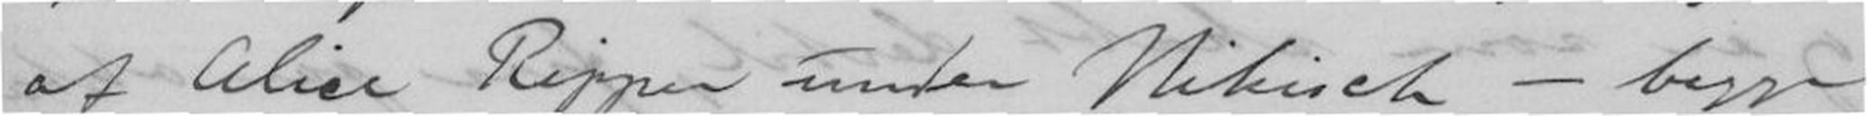af Alice Ripper under Nikisch, - begge
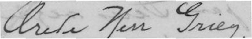Ærede Herr Grieg!
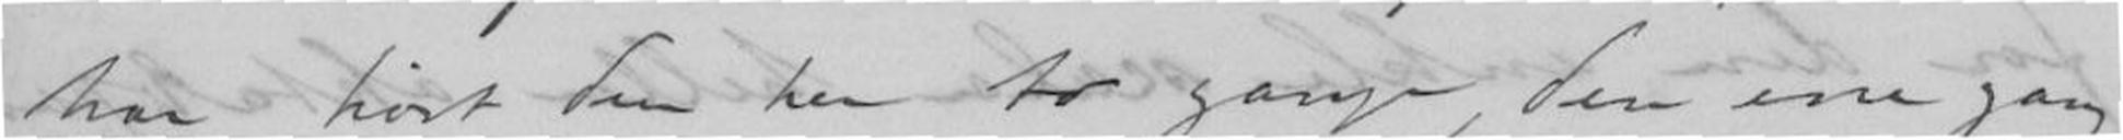har hørt den her to gange, den ene gang
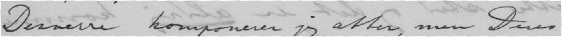Desverre komponerer jeg atter, men Deres
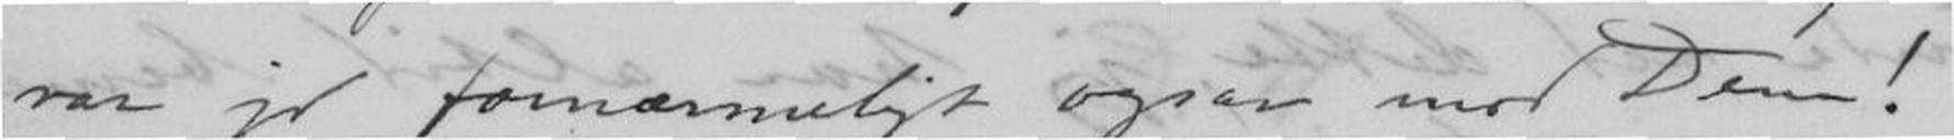var jo fornærmeligt ogsaa mod Dem! -
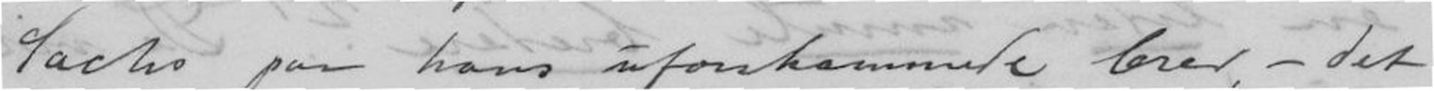Sachs paa hans uforskammede brev, - det
